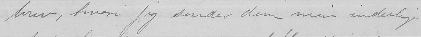brev, hvori jeg sender dem min inderlige
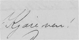Kjære ven!
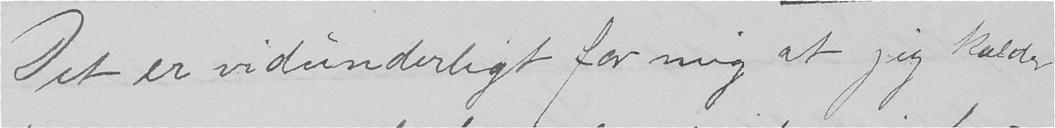Det er vidunderligt for mig at jeg kalder
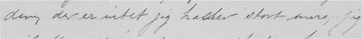dem, der er intet jeg hader stort mere, jeg
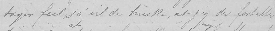tager feil, ja vil de huske, at jeg der fortæller
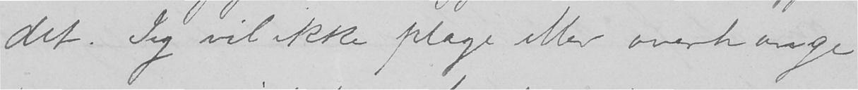det. Jeg vil ikke plage eller overhænge
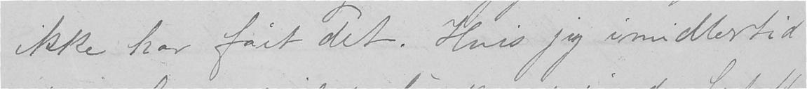ikke har fået det. Hvis jeg imidlertid
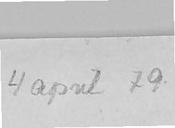4 april 79.
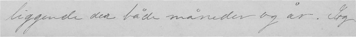liggende der både måneder og år. Jeg
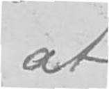at
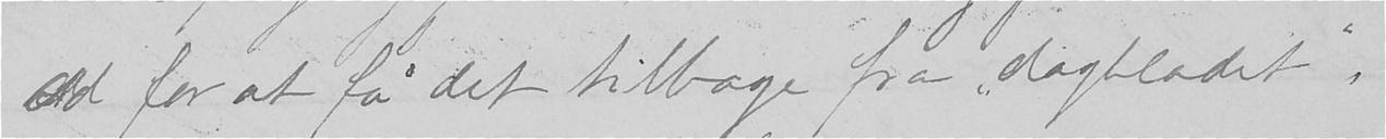ad for at få det tilbage fra "dagbladet",
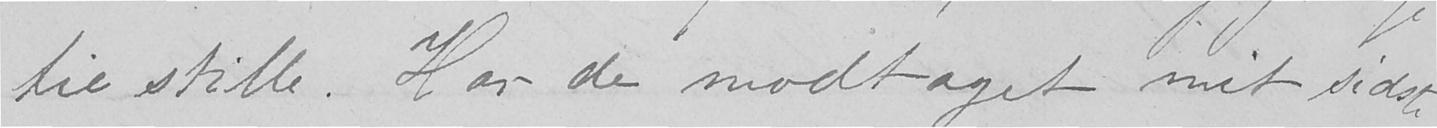tie stille. Har de modtaget mit sidste
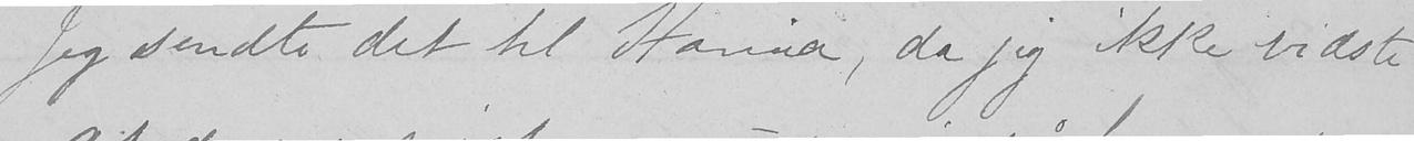Jeg sendte det til Xania, da jeg ikke vidste
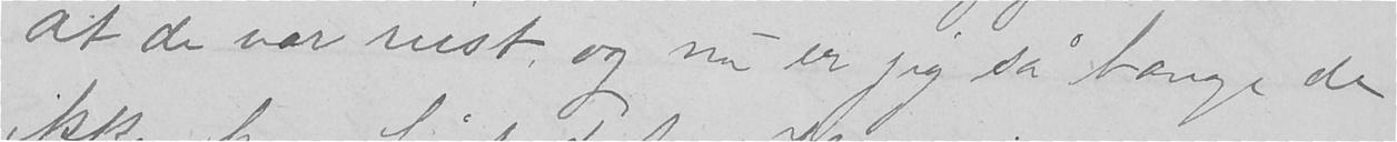at de var reist, og nu er jeg så bange de
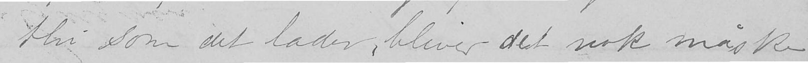thi som det lader, bliver det nok måske
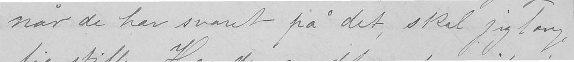når de har svaret på det, skal jeg længe
